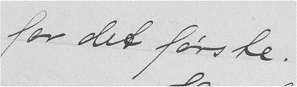for det første.
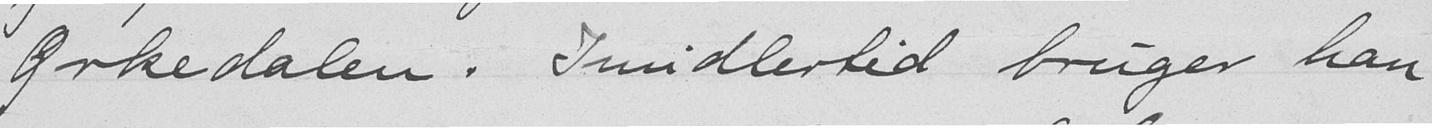Orkedalen. Imidlertid bruger han
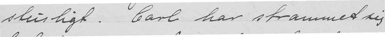stusligt. Carl har strammet sig
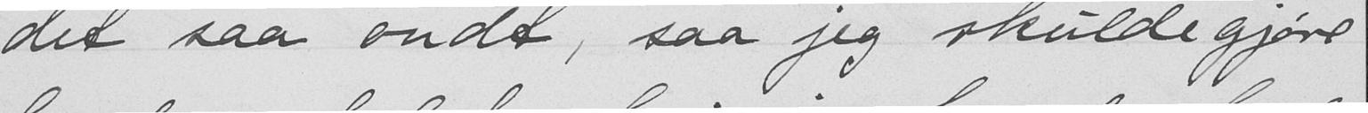det saa ondt, saa jeg skulde gjøre
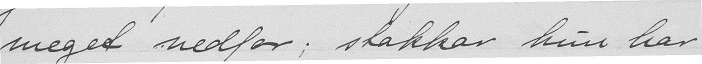meget nedfor; stakkar hun har
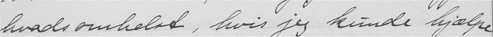hvadsomhelst, hvis jeg kunde hjælpe
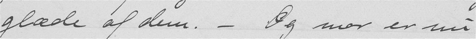glæde af dem. - Og mor er nu
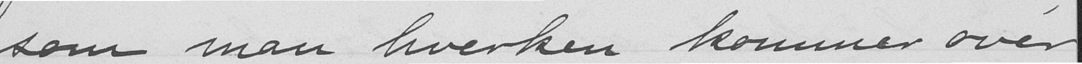som man hverken kommer over
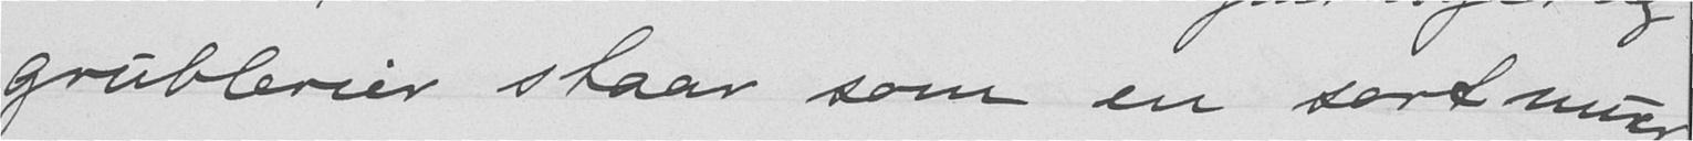grublerier staar som en sort mur,
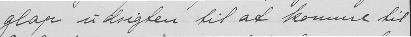glap udsigten til at komme til
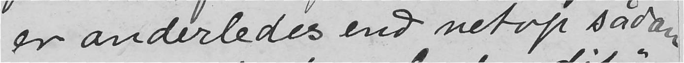er anderledes end netop sådan
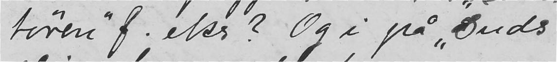tøren" f. eks? Og i på "Guds
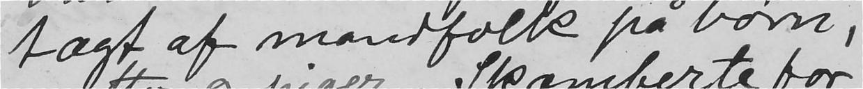tægt af mandfolk på børn,
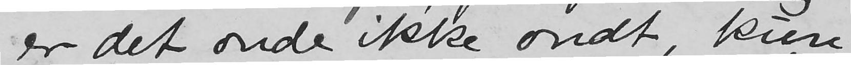er det onde ikke ondt, kun
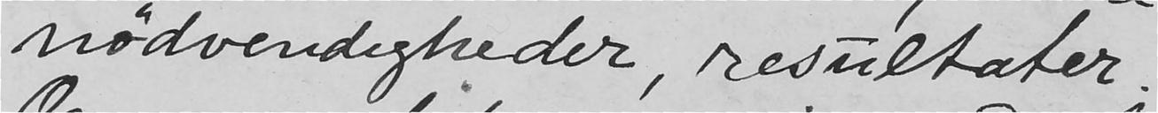nødvendigheder resultater.
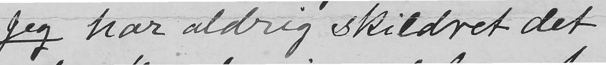Jeg har aldrig skildret det
